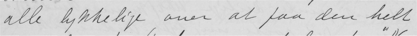alle lykkelige over at faa den helt
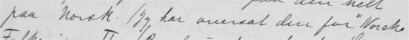paa Norsk. (Jeg har oversat den for "Norske
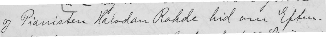og Pianisten Halvdan Rohde hid om Eften.
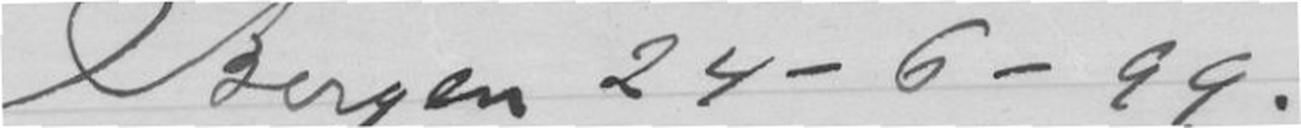Bergen 24-6-99.
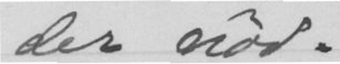der nød.
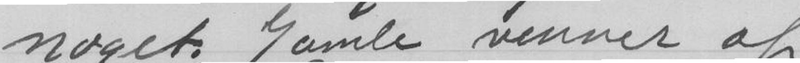noget. Gamle venner af
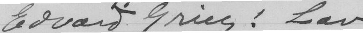Edvard Grieg! Lav
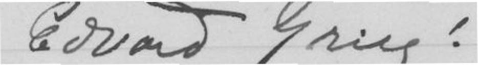Edvard Grieg!
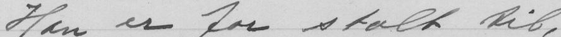Han er for stolt til,
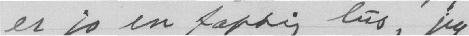er jo en fattig lus, jeg
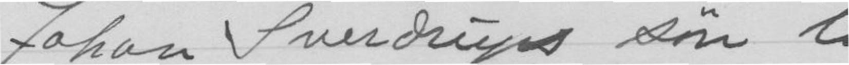Johan Sverdrups søn li-
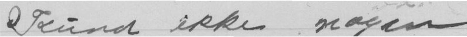Kund ikke nogen
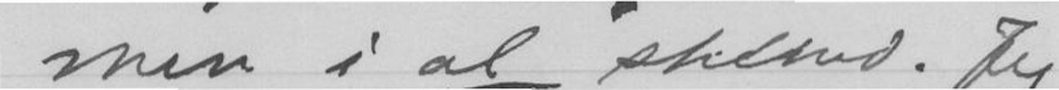men i al stilhed. Jeg
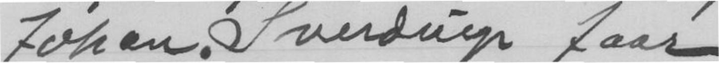Johan Sverdrup faar
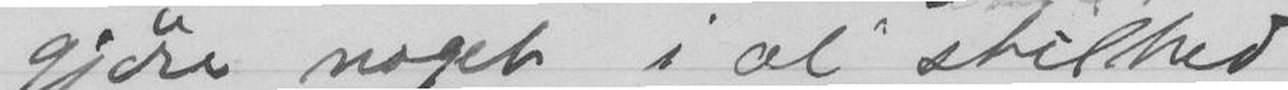gjøre noget i al stilhed
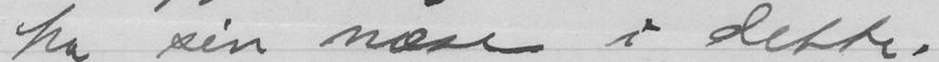ha sin næse i dette.
